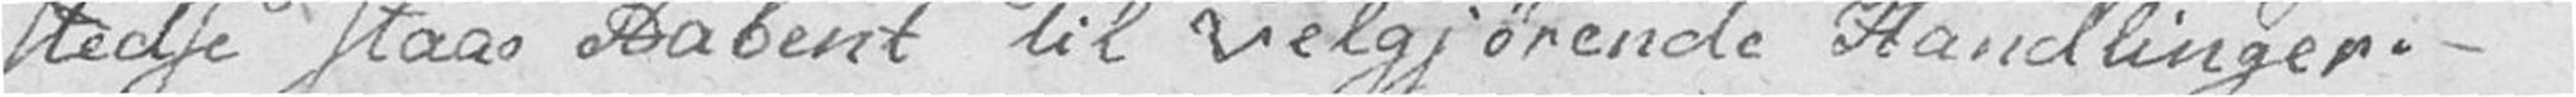stedse staae Aabent til velgjørende Handlinger. –
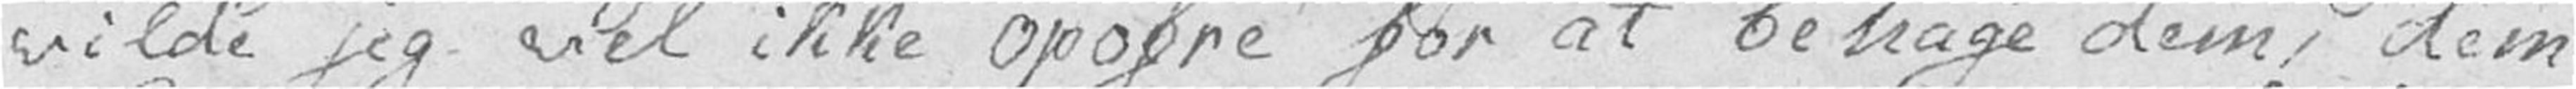vilde jeg vel ikke opofre for at behage dem, dem
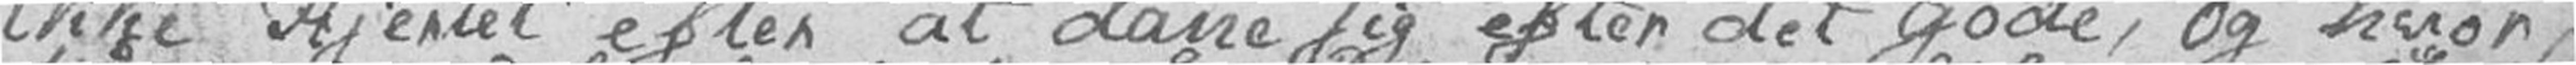ikke Hjertet efter at dane sig efter det gode, og hvor
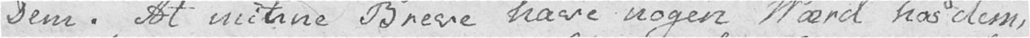Dem. At minne Breve have nogen Værd hos dem,
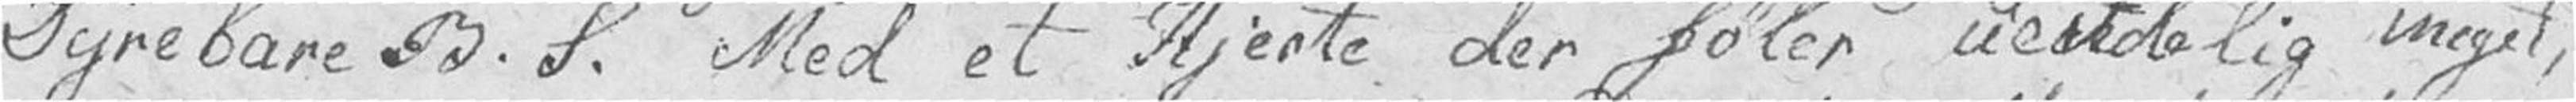Dyrebare B. S. Med et Hjerte der føler uendelig meget,
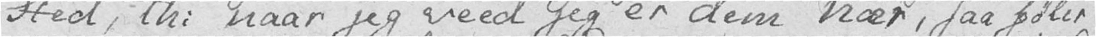Sted, thi naar jeg veed jeg er dem nær, saa føler
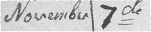November 7de
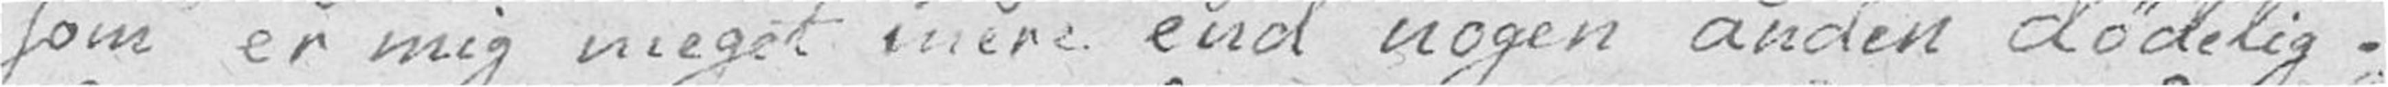som er mig meget mere end nogen anden dødelig.
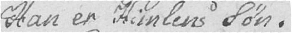Han er Himlens Søn.
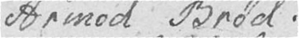Armod Brød!
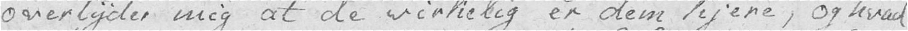overtyder mig at de virkelig er dem kjere, og hvad
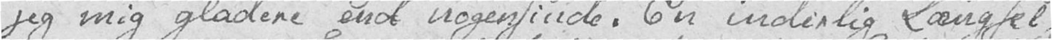jeg mig gladere end nogensinde. En inderlig Længsel
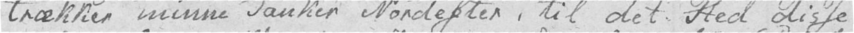trækker minne Tanker Nordefter, til det Sted disse
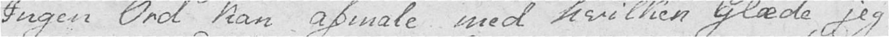Ingen Ord kan afmale med hvilken Glæde jeg
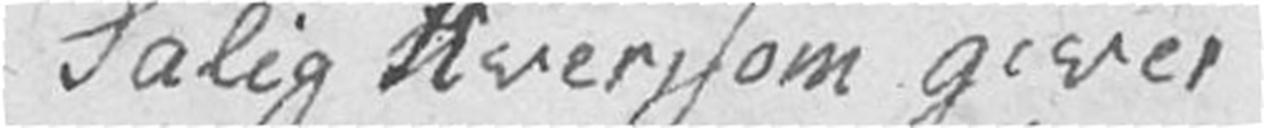Salig Hver, som giver
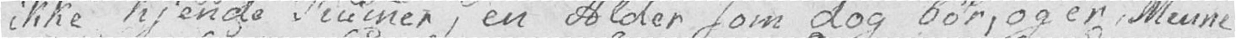ikke Kjende Kummer, en Alder som dog bør, og er, Menne
